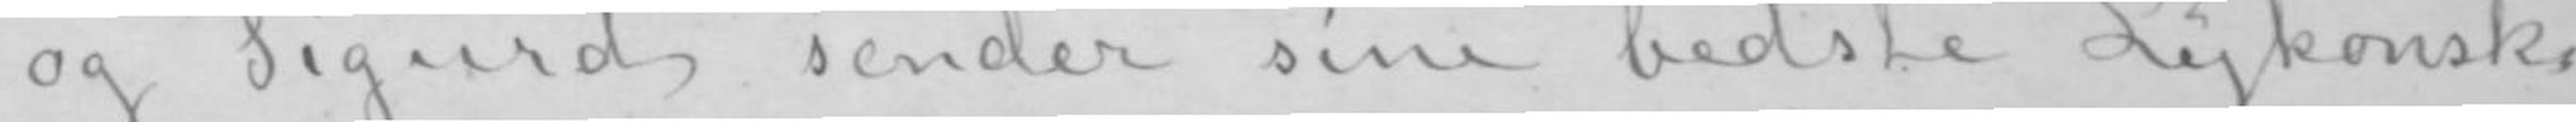og Sigurd sender sine bedste Lykønsk-
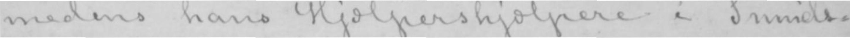medens hans Hjælpershjælpere i Smuds-
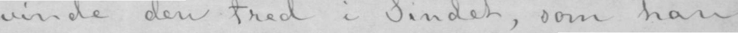vindege den Fred i Sindet, som han
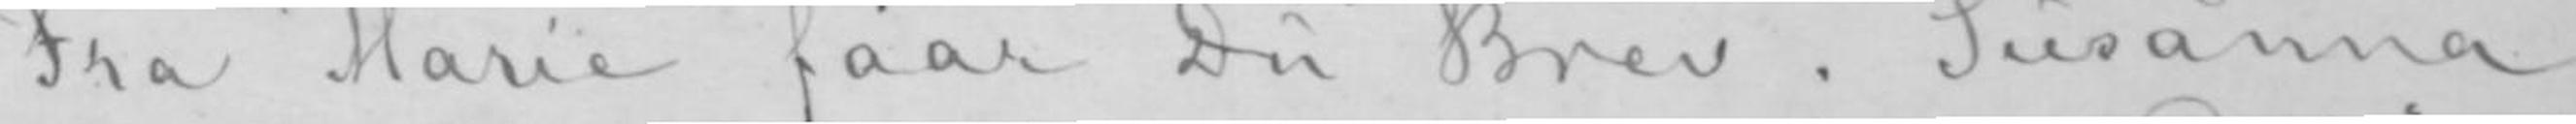Fra Marie faar Du Brev. Susanna
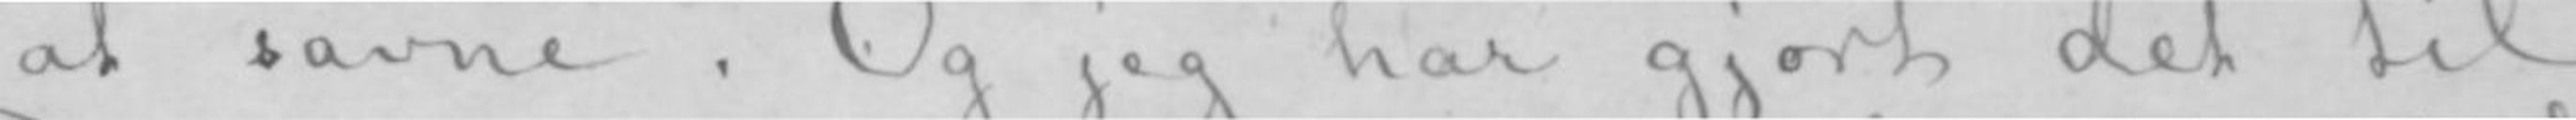at savne. Og jeg har gjort det til
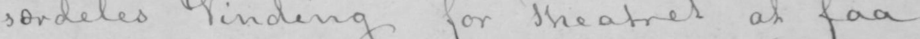særdeles Vinding for Theatret at faa
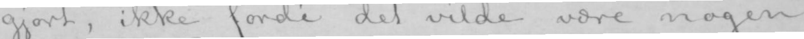gjort, ikke fordi det vilde være nogen
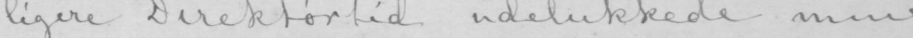ligere Direktørtid udelukkede mine
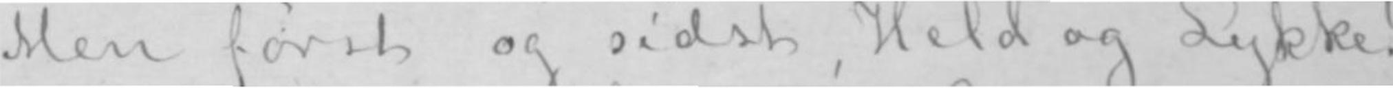Men først og sidst, Held og Lykke!
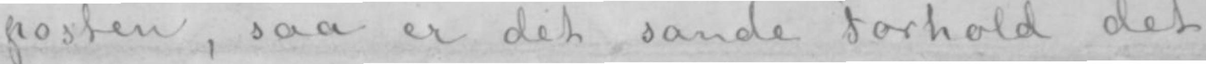posten, saa er det sande Forhold det
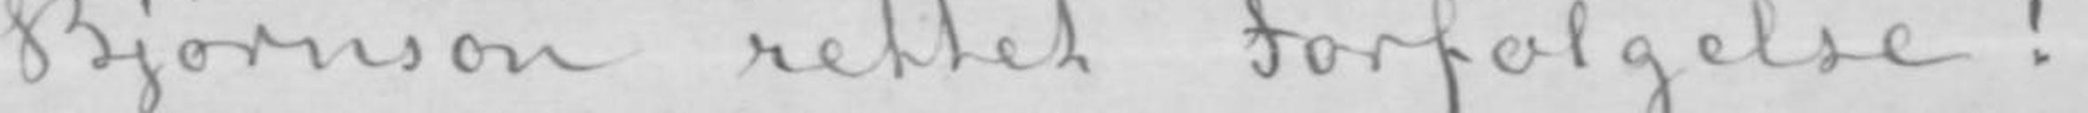Bjørnson rettet Forfølgelse!--
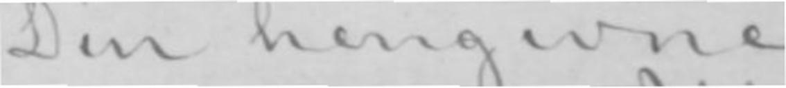Din hengivne
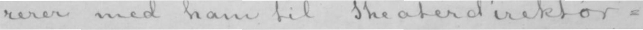rerer med ham til Theaterdirektør-
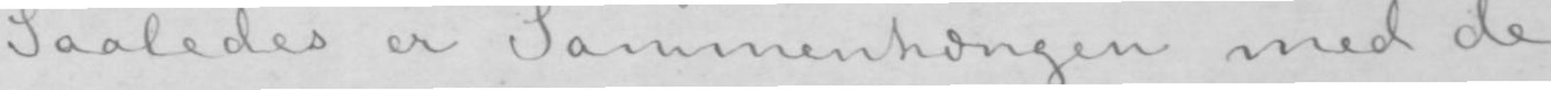Saaledes er Sammenhængen med de
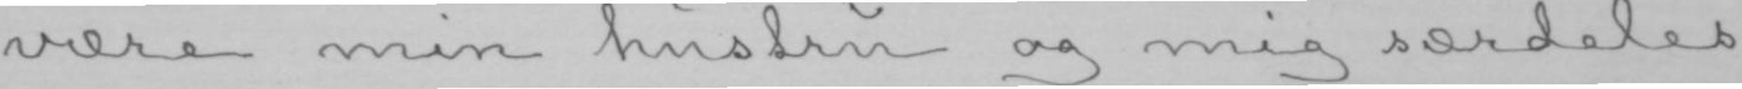være min hustru og mig særdeles
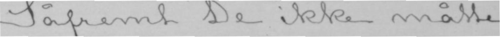Såfremt De ikke måtte
